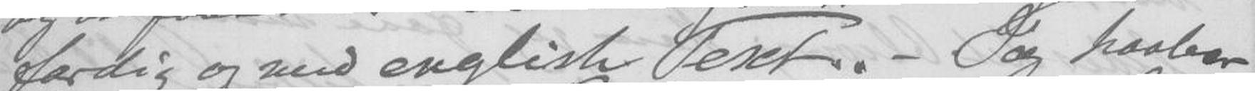færdig og med english Text. - Jeg haaber
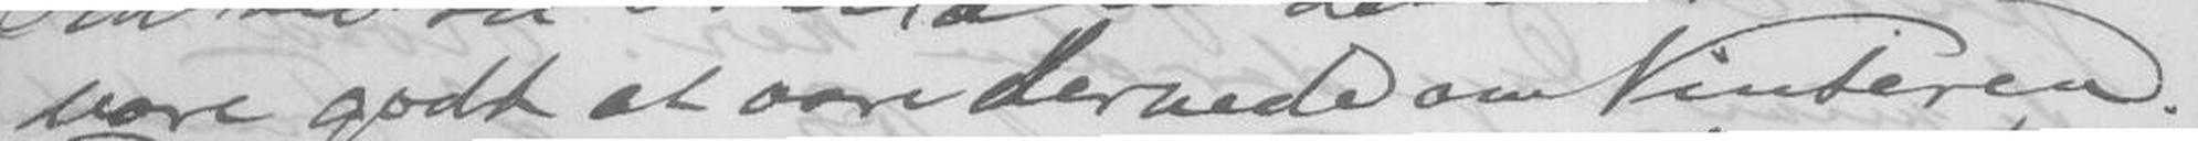være godt at være dernede om Vinteren.
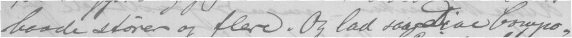baade stører og flere. Og lad saa Dine Compo-
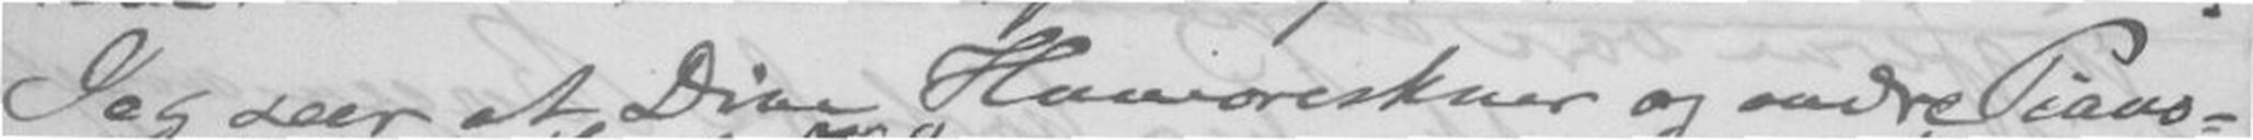Jeg seer at Dine Humoreskner og andre Piano-
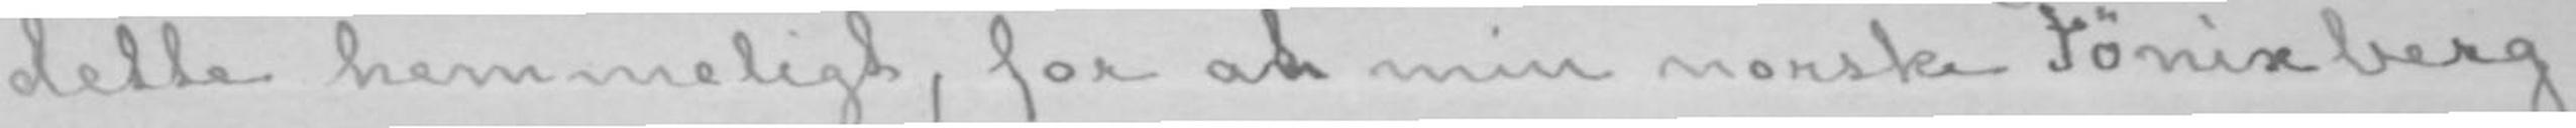dette hemmeligt, for at min norske Fønixberg
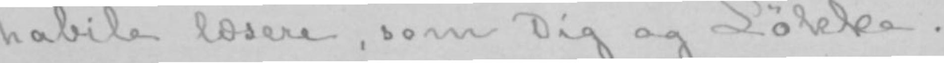habile læsere, som Dig og Løkke.
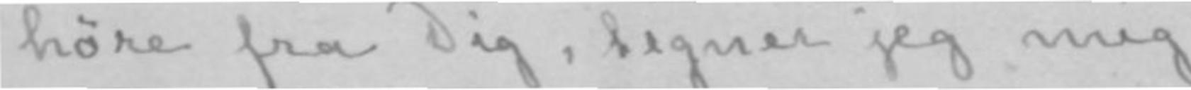høre fra Dig, tegner jeg mig
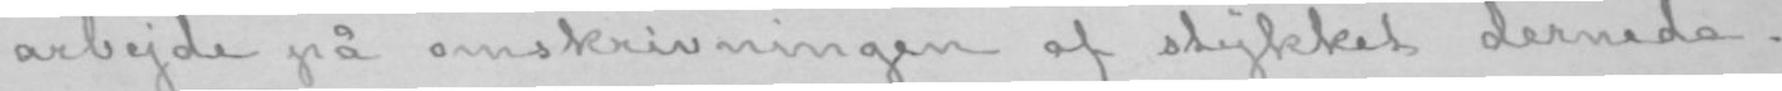arbeide på omskrivningen af stykket dernede.
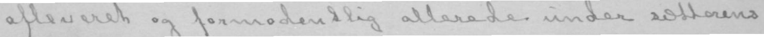afleveret og formodentlig allerede under sætterens
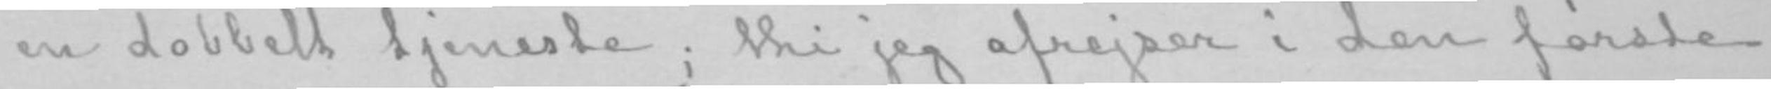en dobbelt tjeneste; thi jeg afrejser i den første
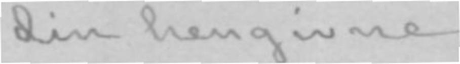din hengivne
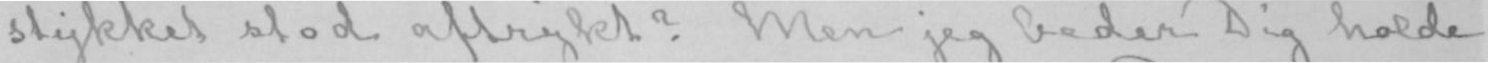stykket stod aftrykt? Men jeg beder Dig holde
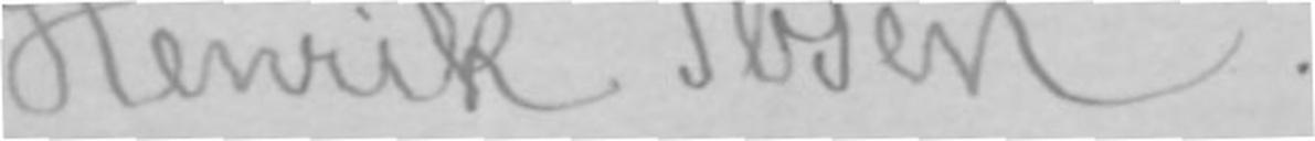Henrik Ibsen.
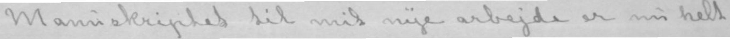Manuskriptet til mit nye arbejde er nu helt
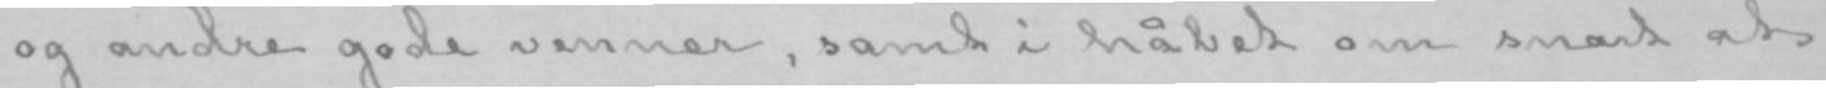og andre gode venner, samt i håbet om snart at
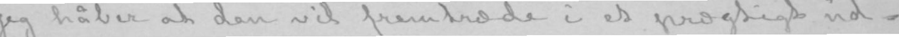jeg håber at den vil fremtræde i et prægtigt ud-
